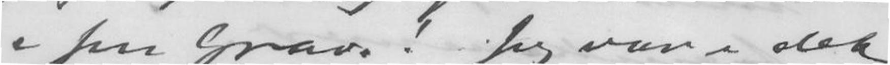i sin Grav! Jeg var i det
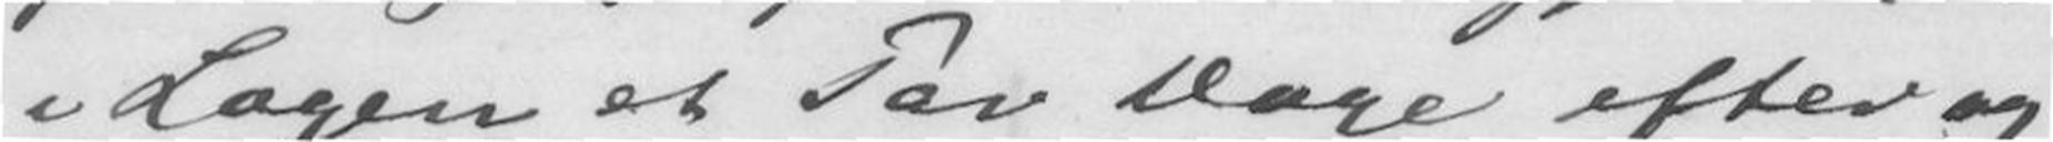i Logen et Par Dage efter og
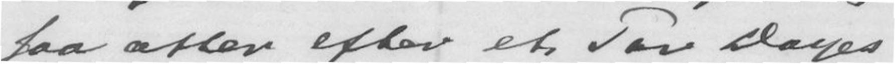saa atter efter et Par Dages
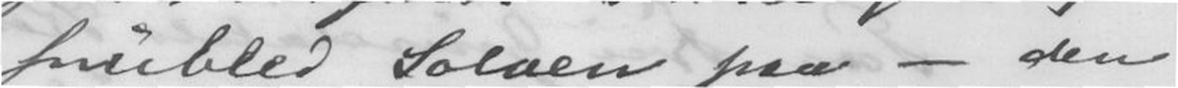snubled Soloen paa - den
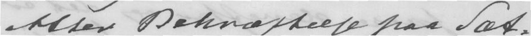Atter Bekræftelse paa Sæt-
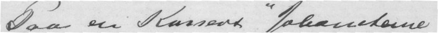Paa en Konsert "Johaniterne"
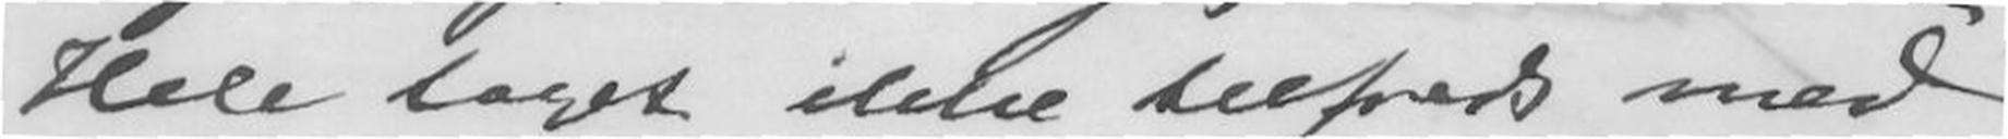Hele taget ikke tilfreds med
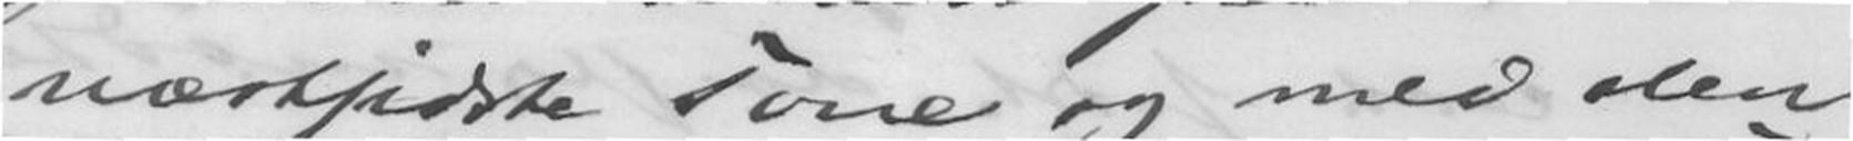næstsidste Tone og med den
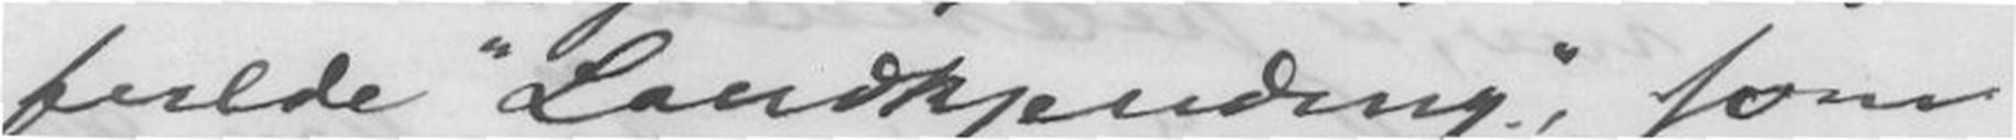fulde "Landkjending", som
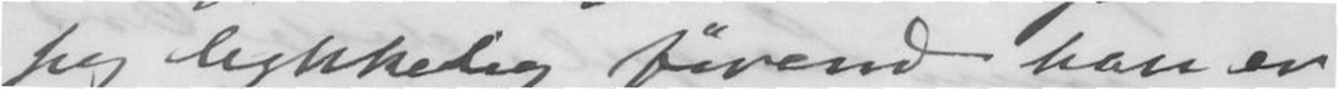sig lykkelig førend han er
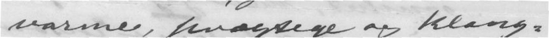varme, prægtige og klang-
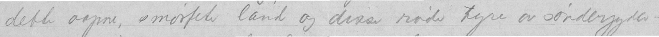dette aapne, smørfete land og disse røde tyre av sønderjyder –
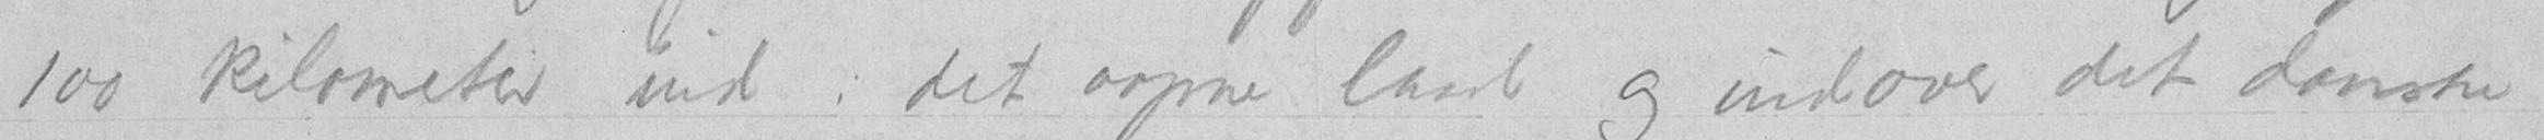100 kilometer ind i det aapne land og indover det danske
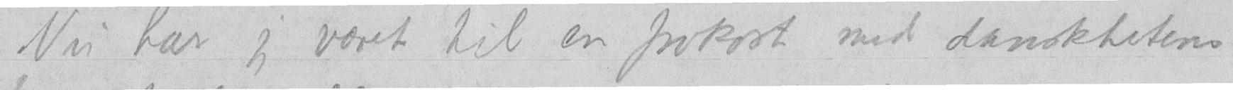Nu har jeg været til en frokost med danskhetens
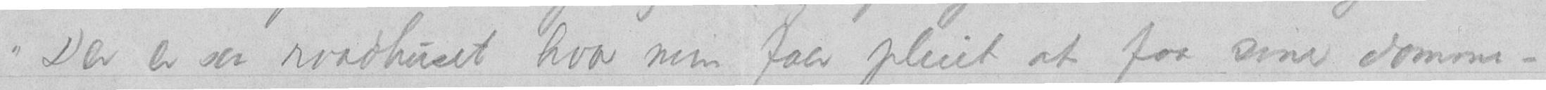"Der er saa raadhuset hvor min faer pleiet at faa sine domme –
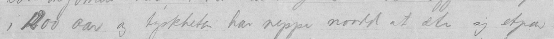i 1200 aar og tyskheten har neppe naad at æte sig etpar
